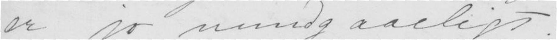er jo uundgaaeligt.
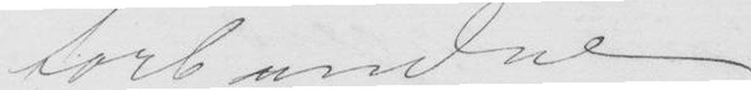forbundne
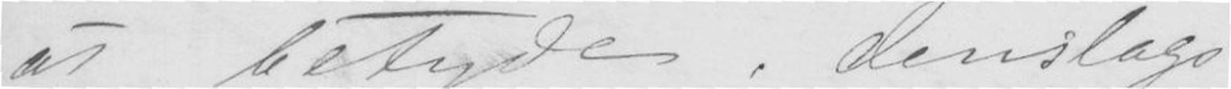at betyde, denslags
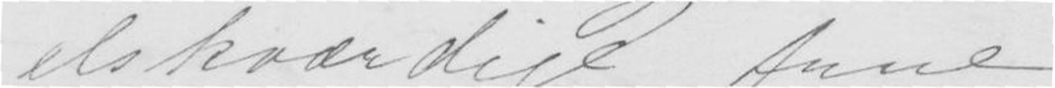elskværdige frue
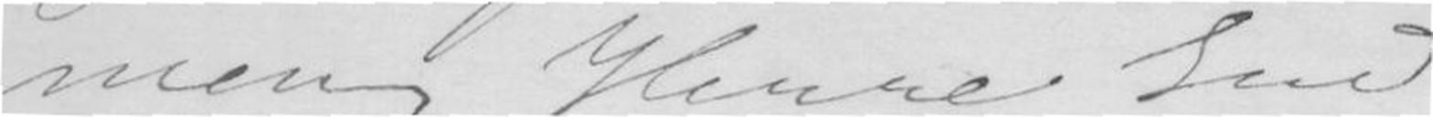men Herre Gud,
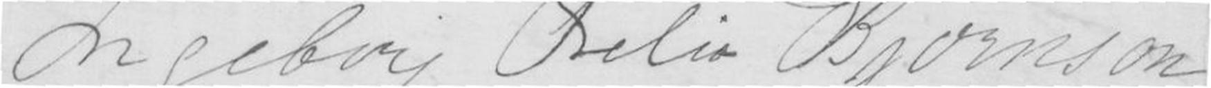Ingeborg Oselio Bjørnson
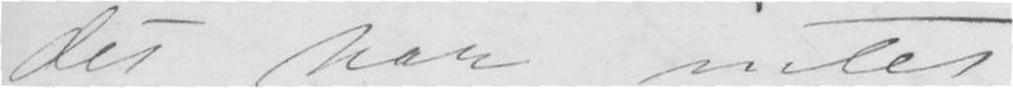det har intet
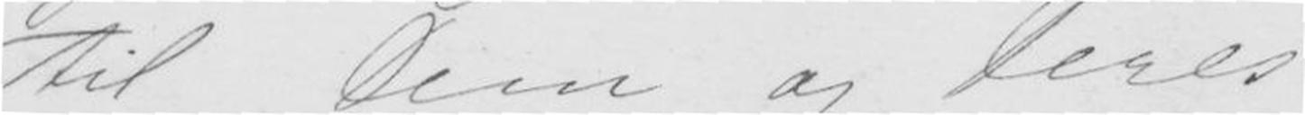til Dem og Deres
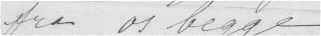fra os begge
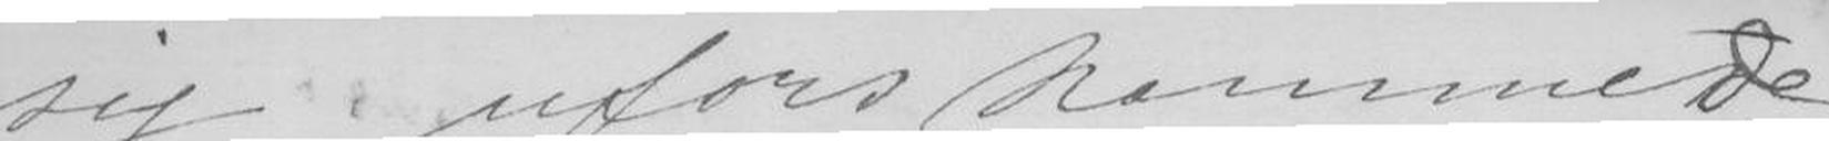sig uforskammende,
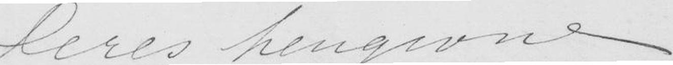Deres hengivne
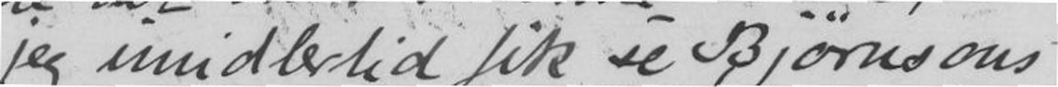jeg imidlertid fik se Bjørnsons
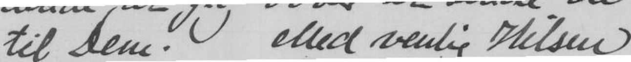til Dem. Med venlig Hilsen
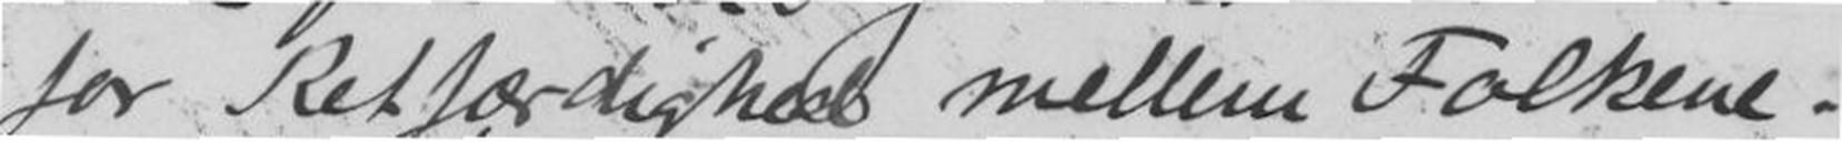for Retfærdighed mellem Folkene.
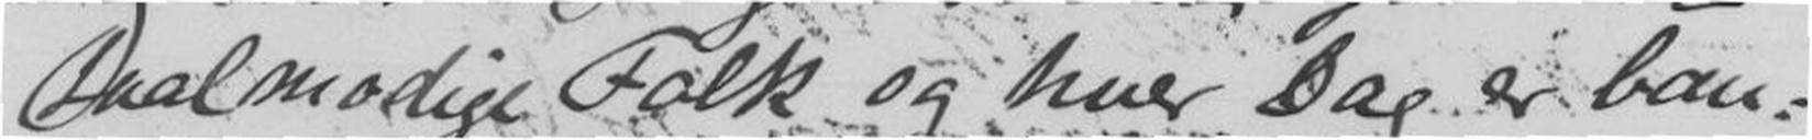taalmodige Folk og hver Dag er ban=
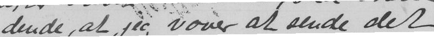dende, at jeg vover at sende det
